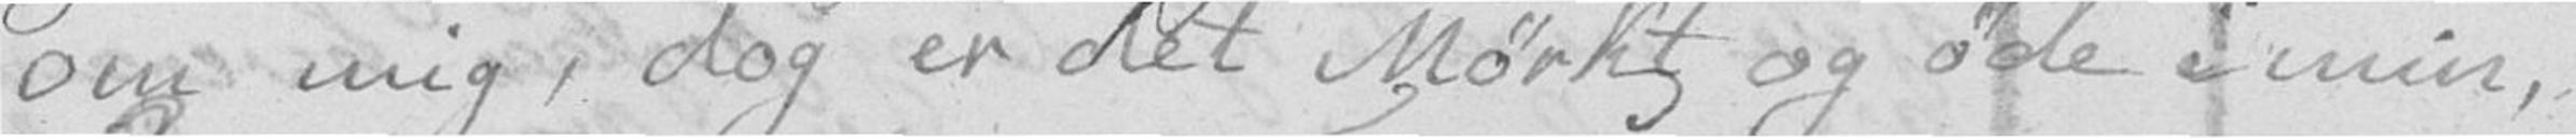om mig, dog er det Mørkt og øde i min,
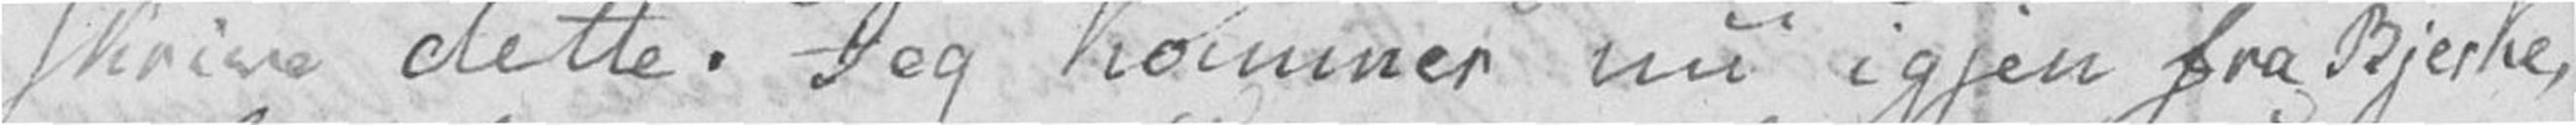skrive dette. Jeg kommer nu igjen fra Bjerke,
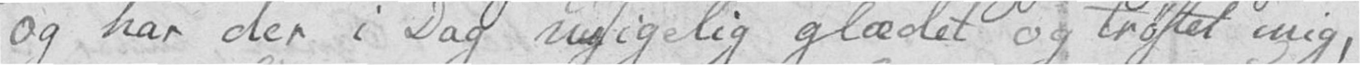og har der i Dag usigelig glædet og trøstet mig,
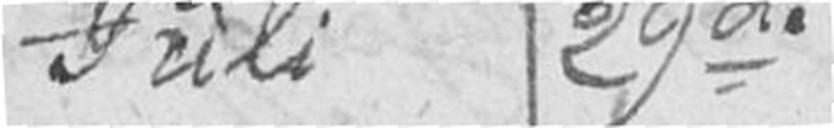Juli 29de
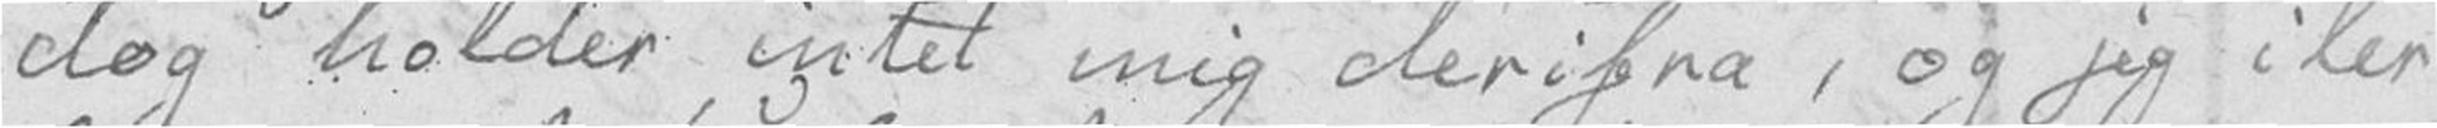dog holder intet mig derifra, og jeg iler
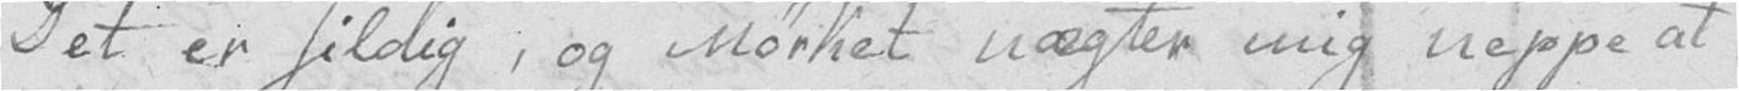Det er sildig, og Mørket nægter mig neppe at
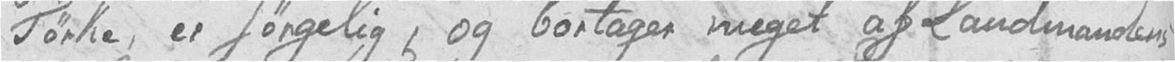Tørke, er sørgelig, og bortager meget af Landmandens
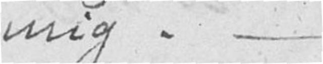mig. –
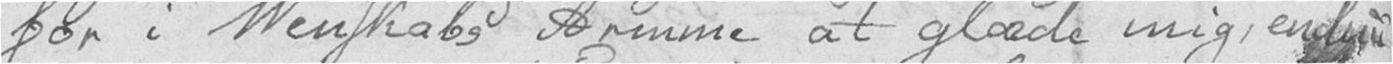for i Venskabs Armme at glæde mig, endnu
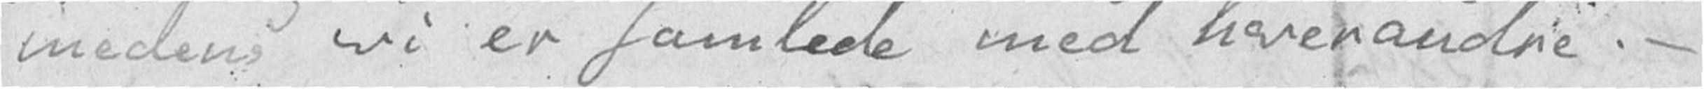medens vi er samlede med hverandre. –
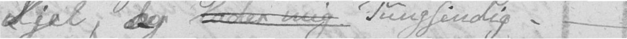Sjel, og lader mig Tungsindig. –
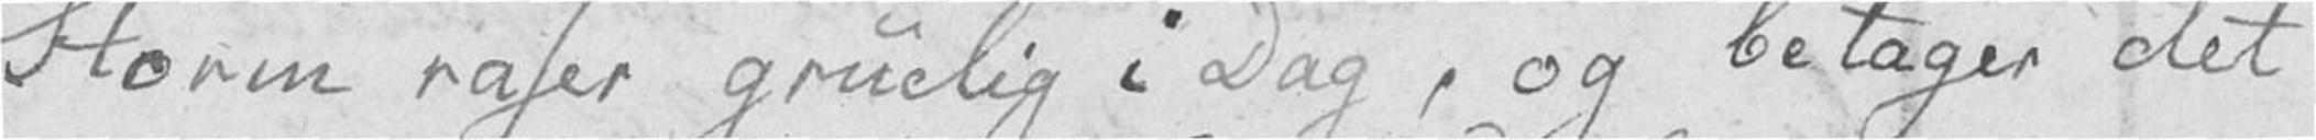Storm raser gruelig i Dag, og betager det
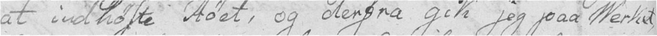at indhøste Høet, og derfra gik jeg paa Verket,
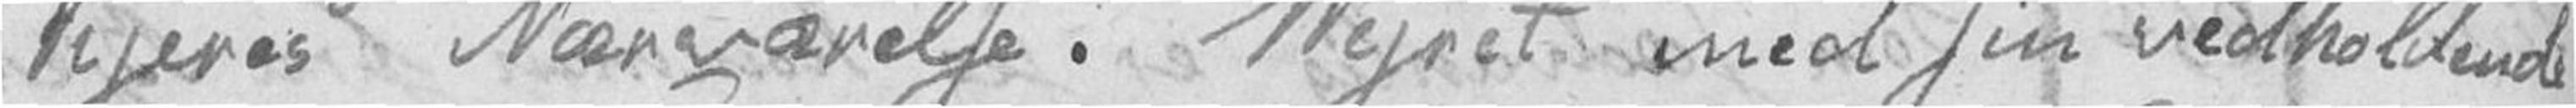Kjeres Nærværelse. Vejret med sin vedholdende
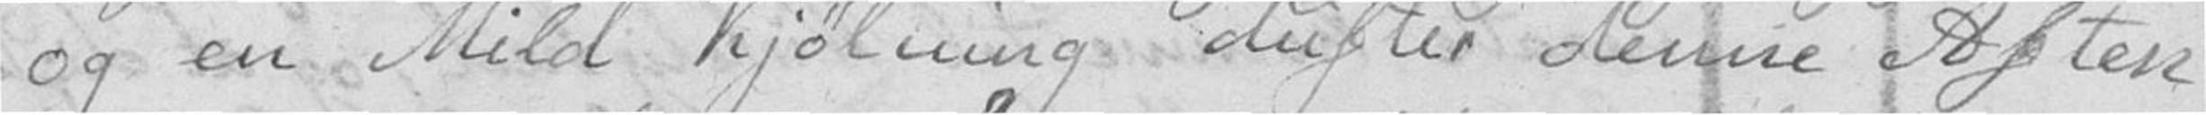og en Mild kjølning dufter denne Aften
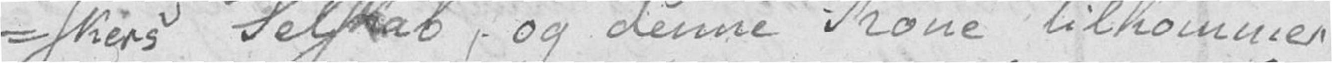-skers Selskab, og denne Kone tilkommer
